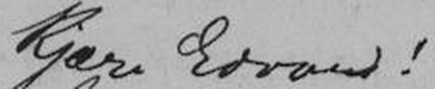Kjære Edvard!
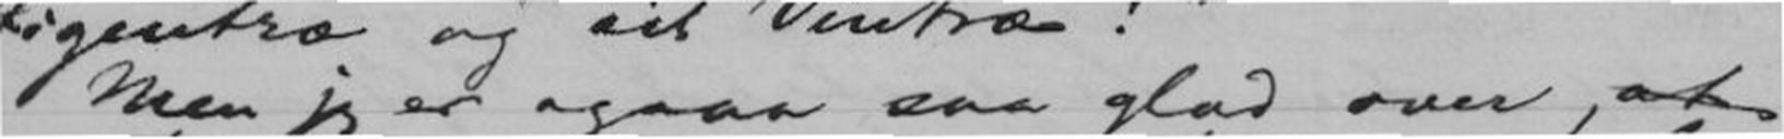Men jeg er ogsaa saa glad over, at
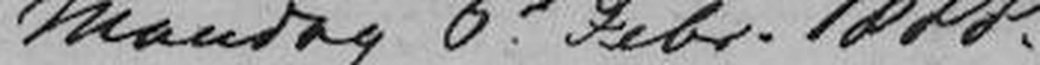Mandag 6 Febr. 1888.
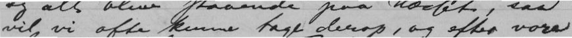vil vi ofte kunne tage derop, og efter vore
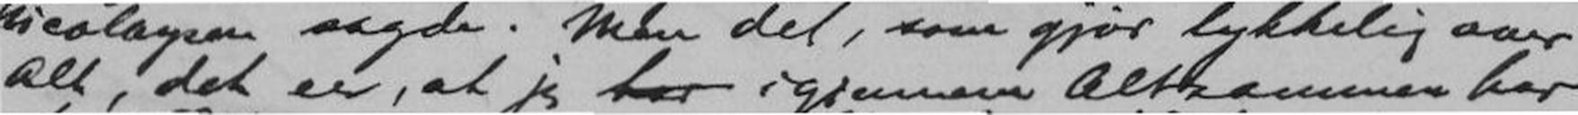Alt, det er, at jeg har igjennem Altsammen har
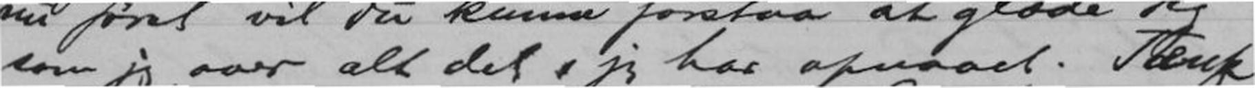som jeg over alt det, jeg har opnaaet. Tænk
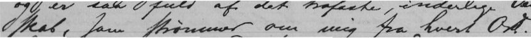skab, som strømmer om mig fra hvert Ord.
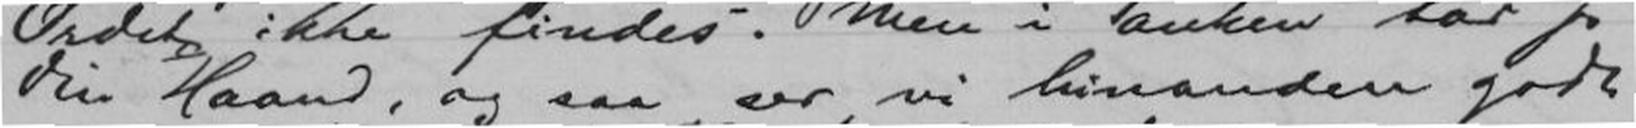din Haand, og saa ser vi hinanden godt
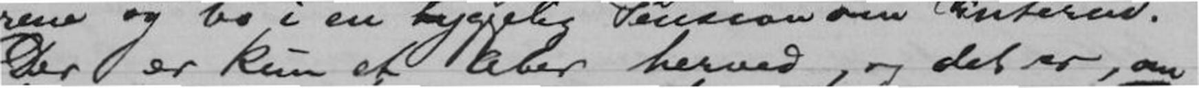Der er kun et Aber herved og det er, om
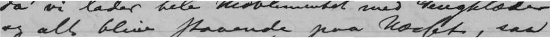og alt blive staaende paa Næsset, saa
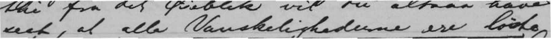seet, at alle Vanskelighederne ere løste
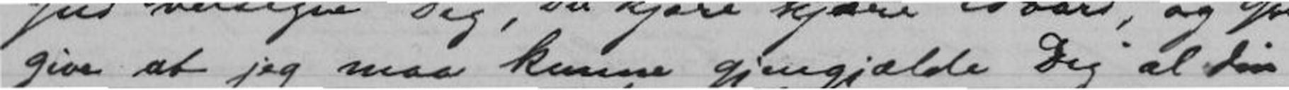give at jeg maa kunne gjengjælde Dig al din
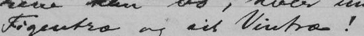Figentræ og sit Vintræ!"
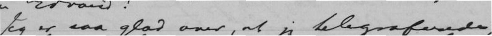Jeg er saa glad over, at jeg telegraferede,
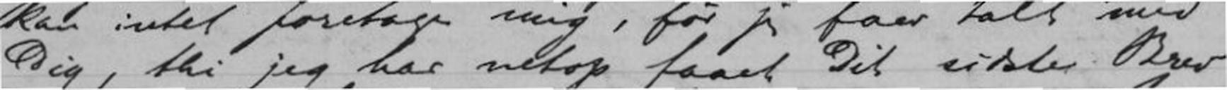Dig, thi jeg har netop faaet Dit sidste Brev
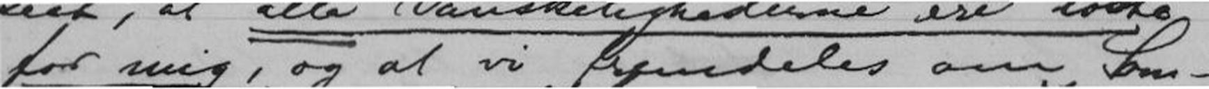for mig, og at vi fremdeles om Som-
